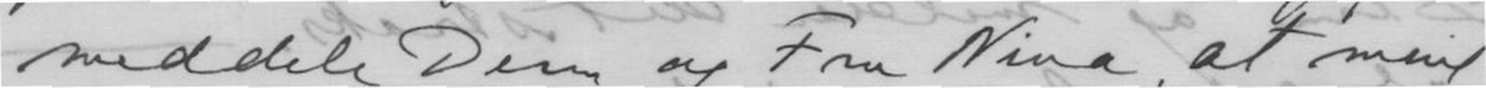meddele Dem og Fru Nina, at mine
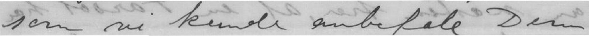som vi kunde anbefale Dem.
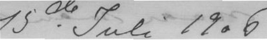15de Juli 1906
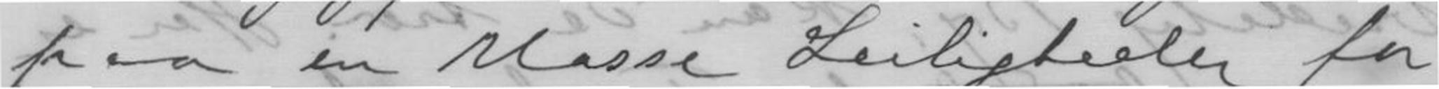paa en Masse Leiligheder for
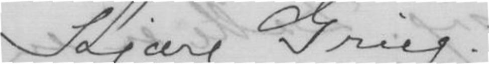Kjære Grieg!
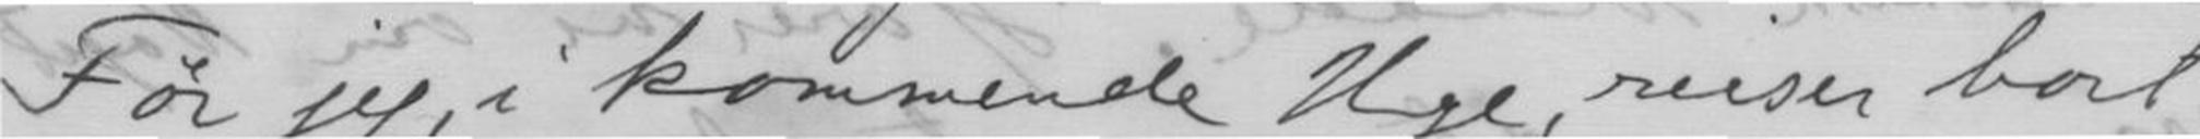Før jeg, i kommende Uge, reiser bort
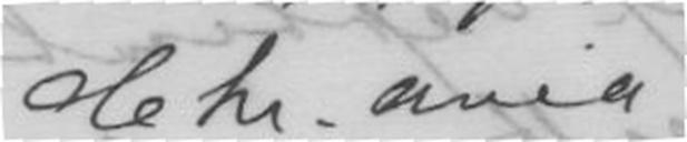Chr.ania
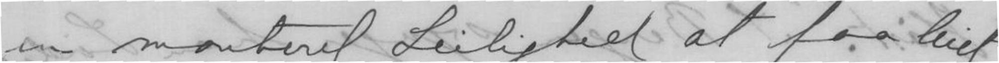en monteret Leilighed at faa leiet
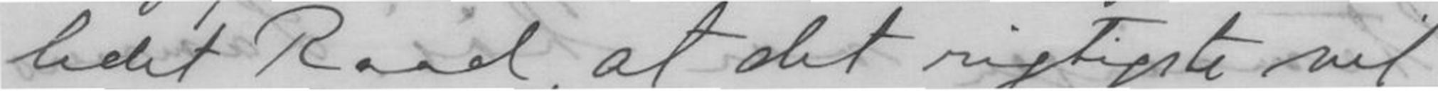lidet Raad, at det rigtigste vil
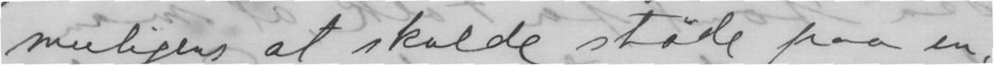muligens, at skulde støde paa en
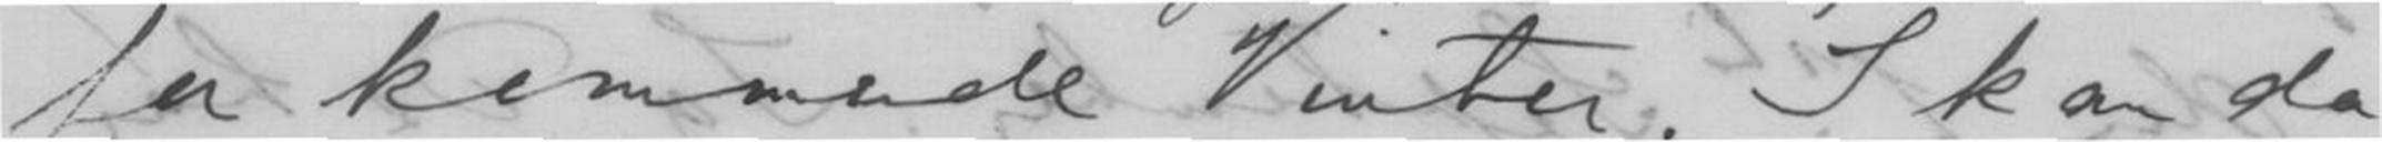for kommende Vinter. I kan da
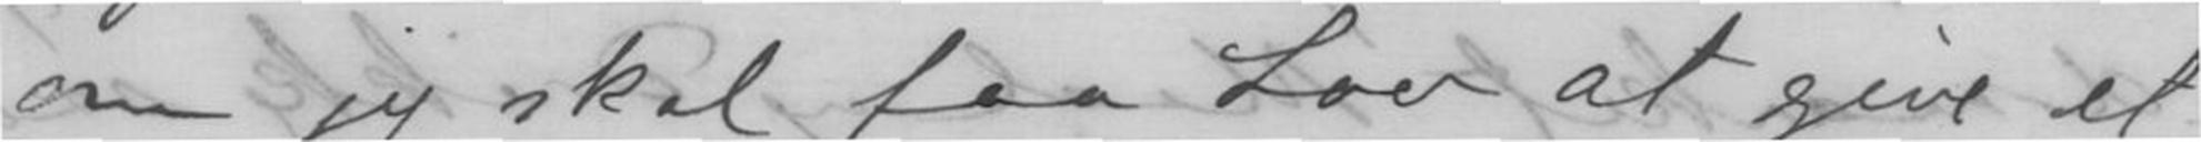om jeg skal faa Lov at give et
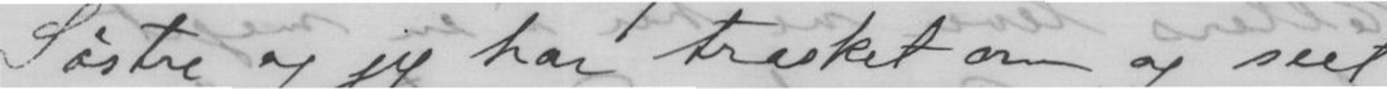Søstre og jeg har trasket om og seet
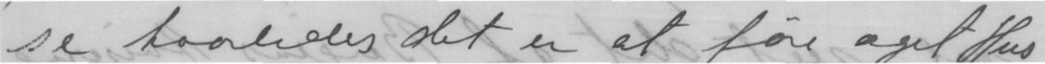se hvorledes det er at føre eget Hus
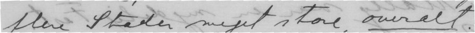flere Stader meget store, overalt.
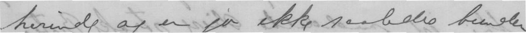herinde og er jo ikke saaledes bunden,
